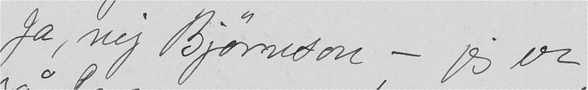Ja, nej Bjørnson - jeg er
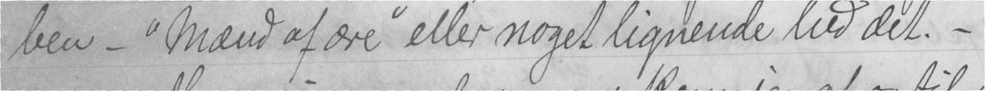ben - "Mænd af ære" eller noget lignende hed det. -
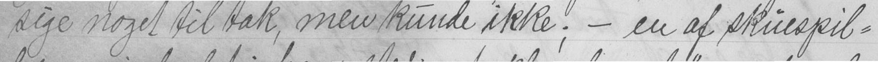sige noget til tak, men kunde ikke; - en af skuespil-
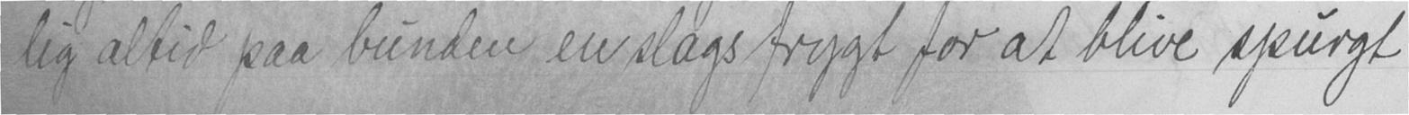lig altid paa bunden en slags frygt for at blive spurgt
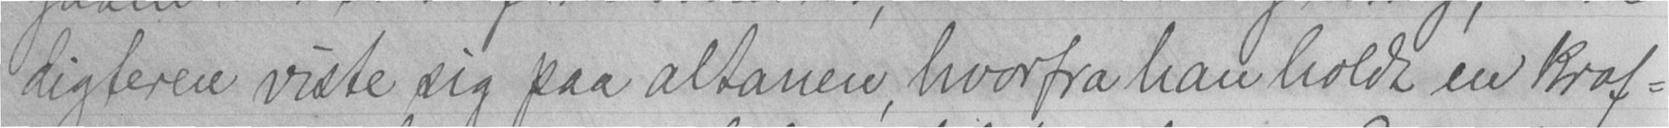digteren viste sig paa altanen, hvorfra han holdt en kraf-
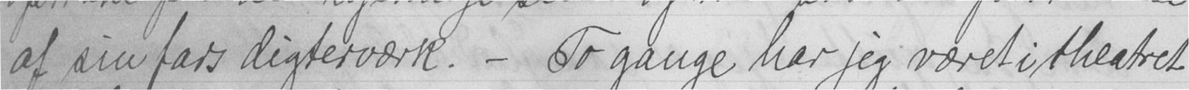af sin fars digterværk. - To gange har jeg været i theatret
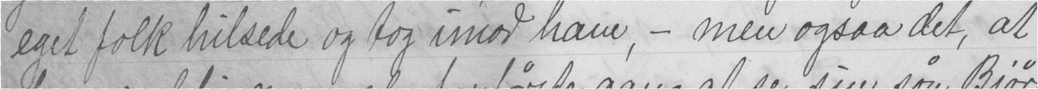eget folk hilsede og tog imod ham, - men ogsaa det, at
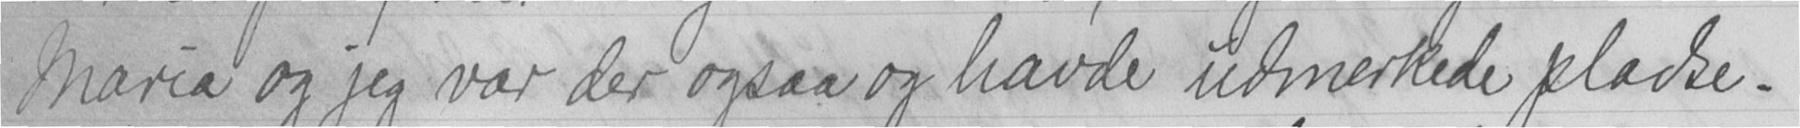Maria og jeg var der ogsaa og havde udmærkede pladse.
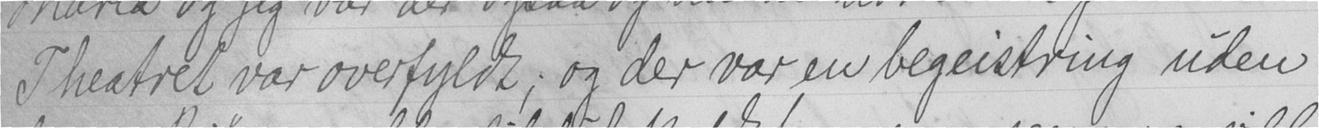Theatret var overfyldt; og der var en begeistring uden
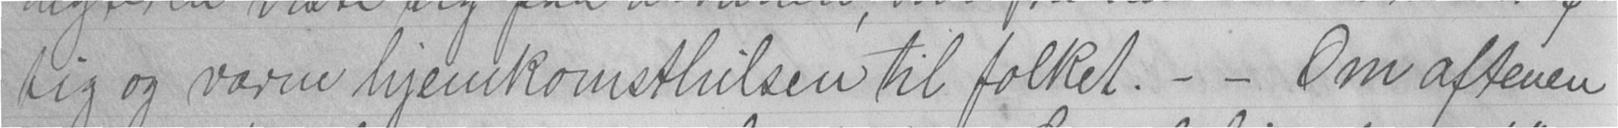tig og varm hjemkomsthilsen til folket. - - Om aftenen
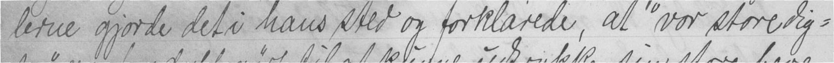lerne gjorde det i hans sted og forklarede, at "vor store dig-
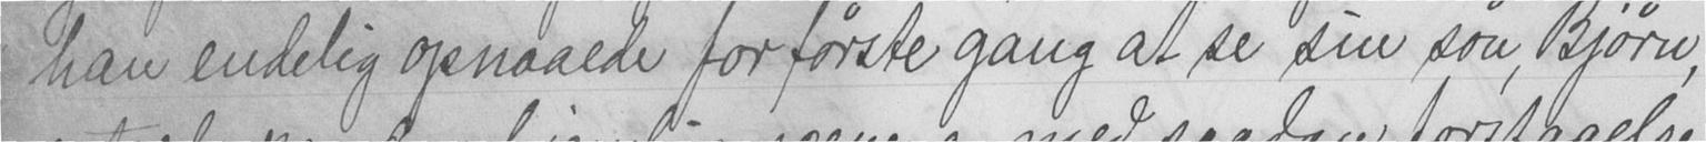han endelig opnaaede for første gang at se sin søn, Bjørn,
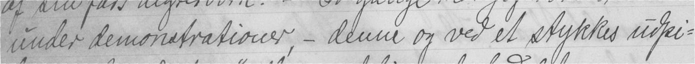under demonstrationer, - denne og ved et stykkes udpi-
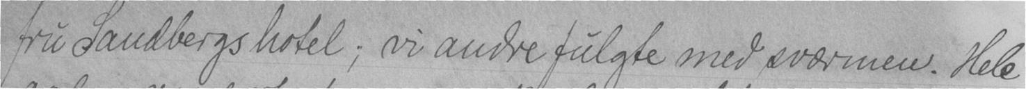fru Sandbergs hotel; vi andre fulgte med sværmen. Hele
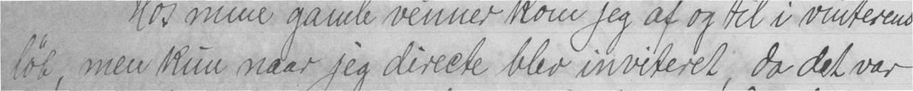løb, men kun naar jeg directe blev inviteret, da det var
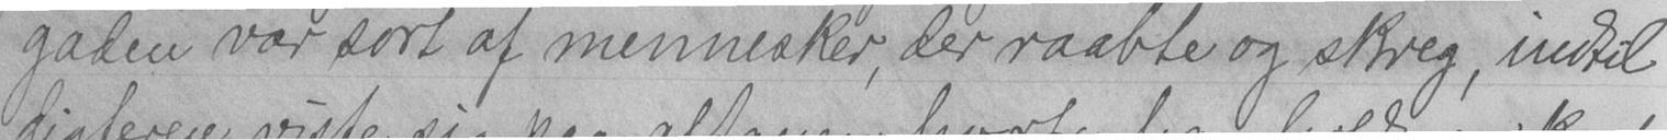gaden var sort af mennesker, der raabte og skreg, indtil
